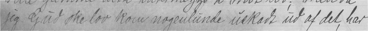jeg Gud ske lov kom nogenlunde uskadt ud af det, har
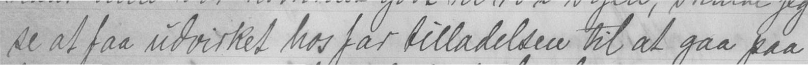se at faa udvirket hos far tilladelsen til at gaa paa
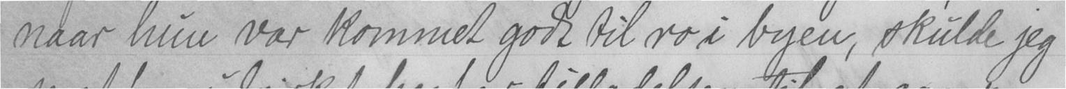naar hun var kommet godt til ro i byen, skulde jeg
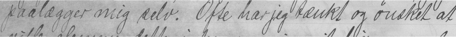paalægger mig selv. Ofte har jeg tænkt og ønsket at
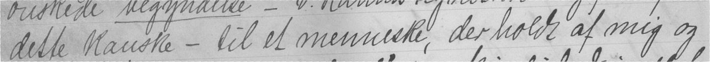dette, kanske - til et menneske, der holdt af mig og
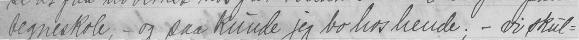tegneskole, - og saa kunde jeg bo hos hende; - vi skul-
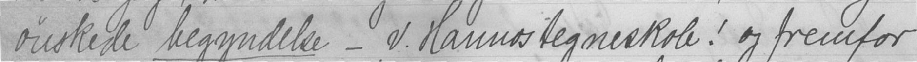ønskede begyndelse - V. Hannos tegneskole! og fremfor
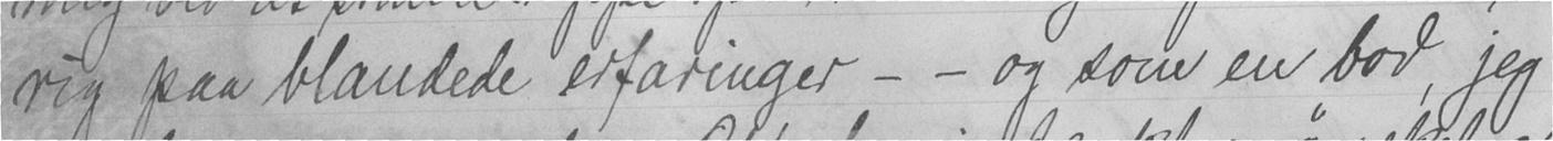rig paa blandede erfaringer - - og som en bod, jeg
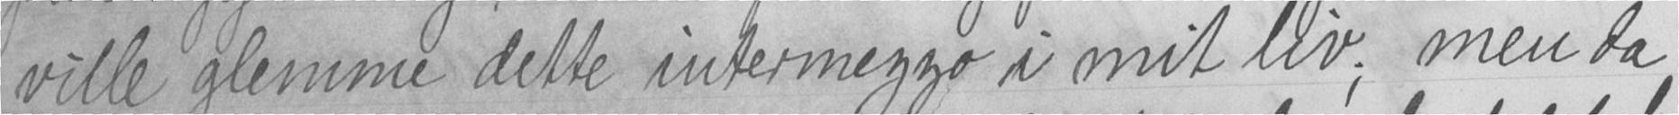ville glemme dette intermezzo i mit liv; men da
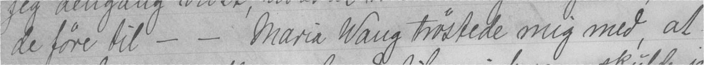de føre til - - Maria Wang trøstede mig med, at
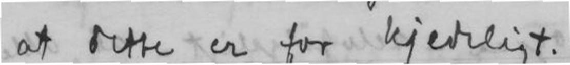at dette er for kjedeligt.
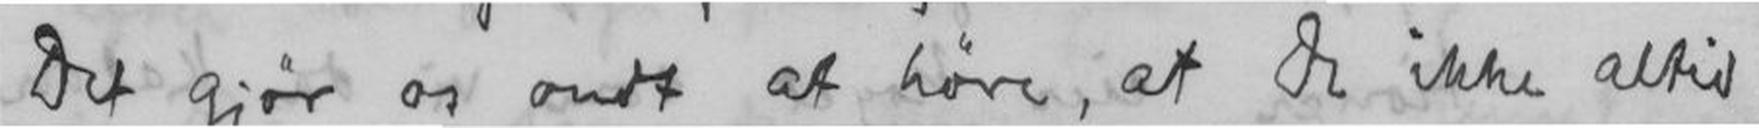Det gjør os ondt at høre, at De ikke altid
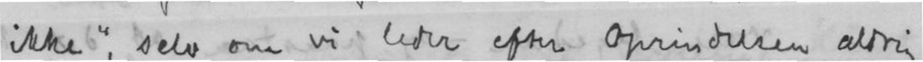ikke, selv om vi leder efter Oprindelsen aldrig
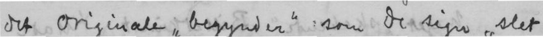det Originale begynder som De siger slet
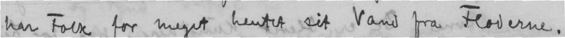har Folk for meget hentet sit Vand fra Floderne.
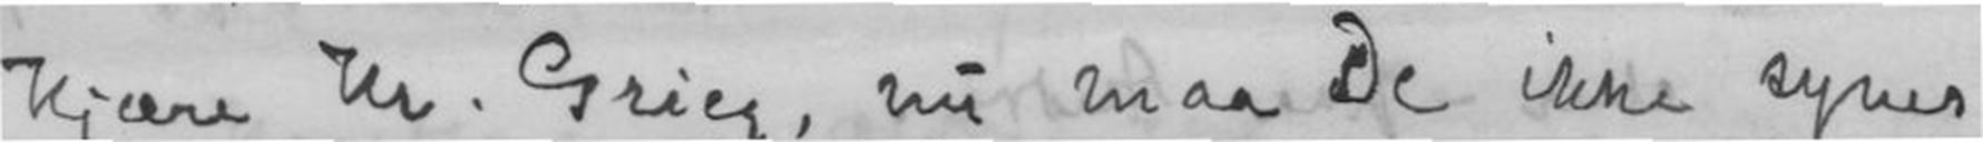Kjære Hr. Grieg, nu maa De ikke synes
